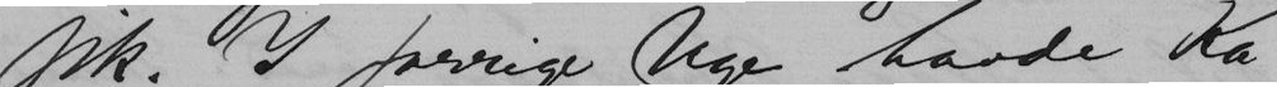ik. I forrige Uge havde Ka-
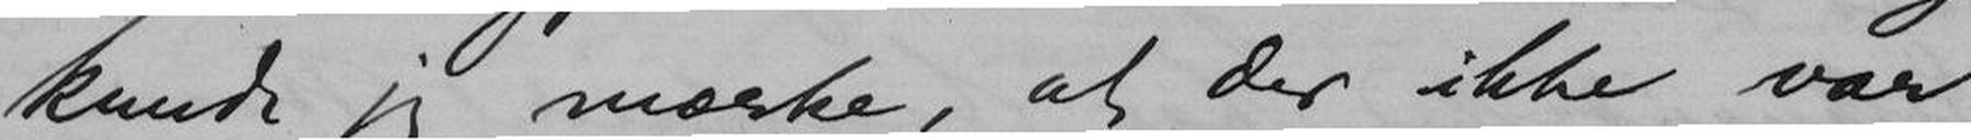kunde jeg mærke, at der ikke var
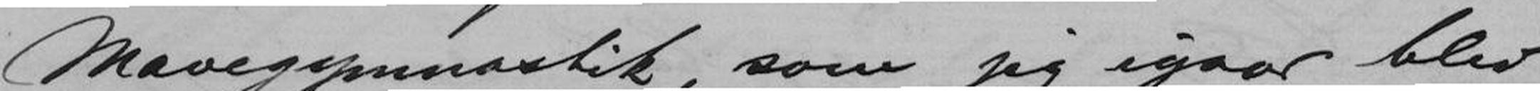Mavegymnastik, som jeg igaar blev
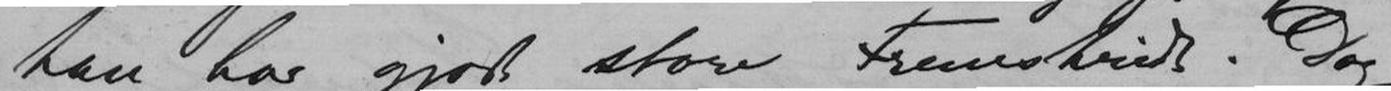han har gjort store Fremskridt. Dog
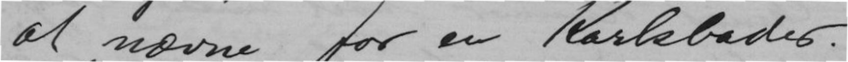at nævne for en Karlsbader.
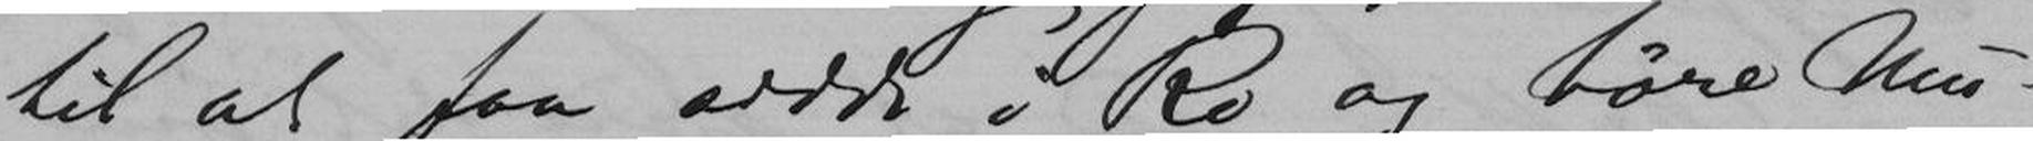til at faa sidde i Ro og høre Mu-
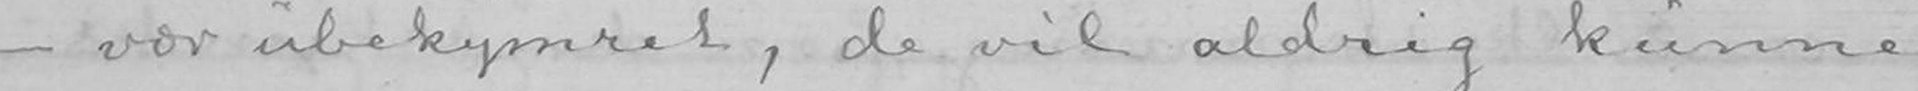- vær ubekymret, de vil aldrig kunne
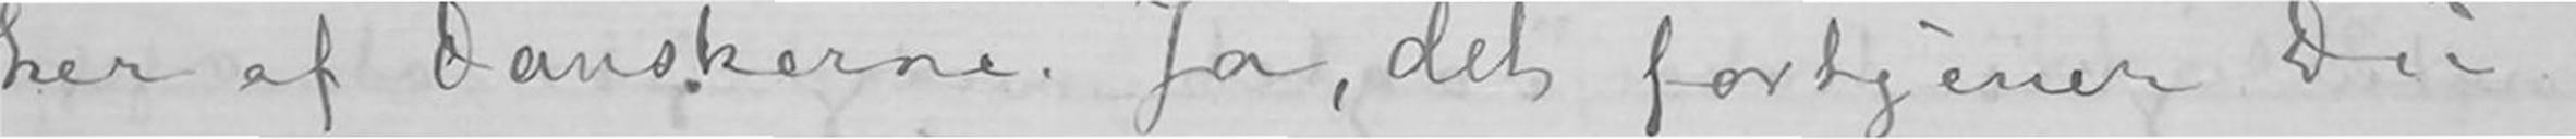her af Danskerne. Ja, det fortjener Du.
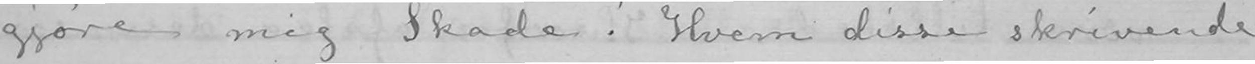gjøre mig Skade! Hvem disse skrivende
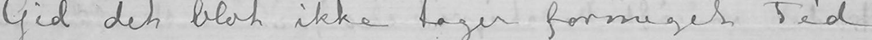Gid det blot ikke tager formeget Tid
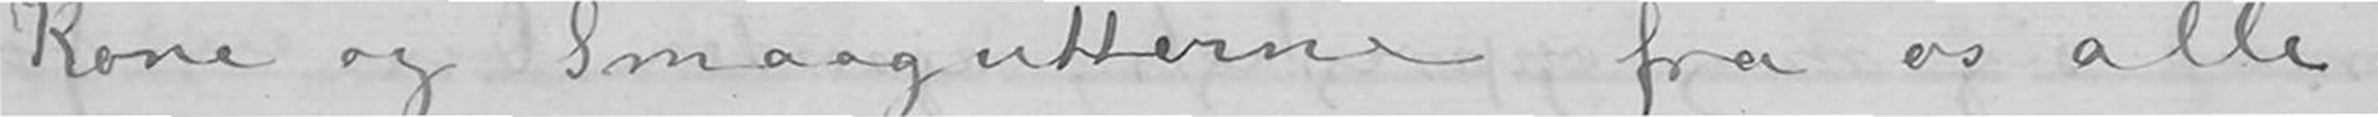Kone og Smaagutterne fra os alle.
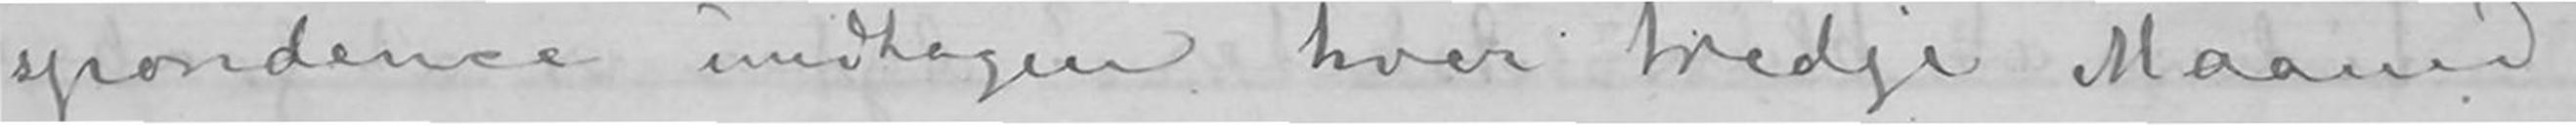spondence undtagen hver tredje Maaned
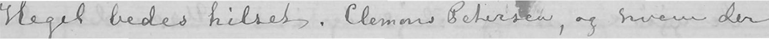Hegel bedes hilset. Clemens Petersen, og hvem der
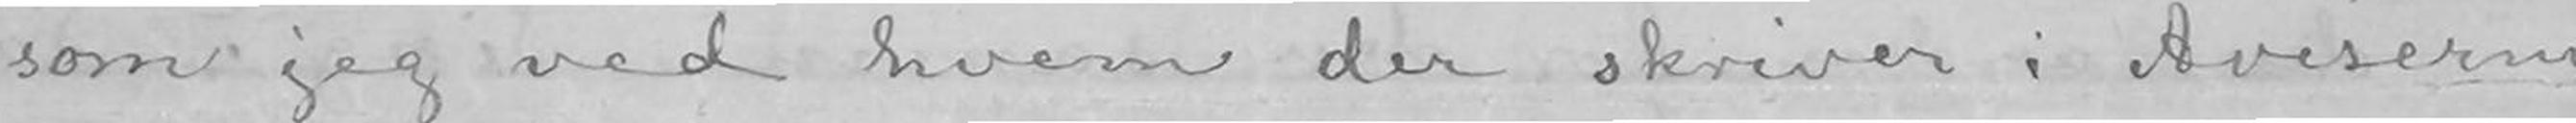som jeg ved hvem der skriver i Aviserne
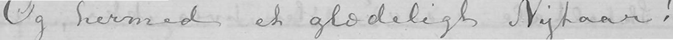Og hermed et glædeligt Nytaar!
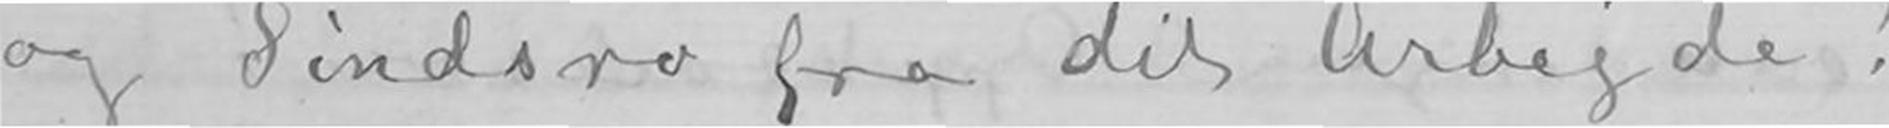og Sindsro fra dit Arbejde!
